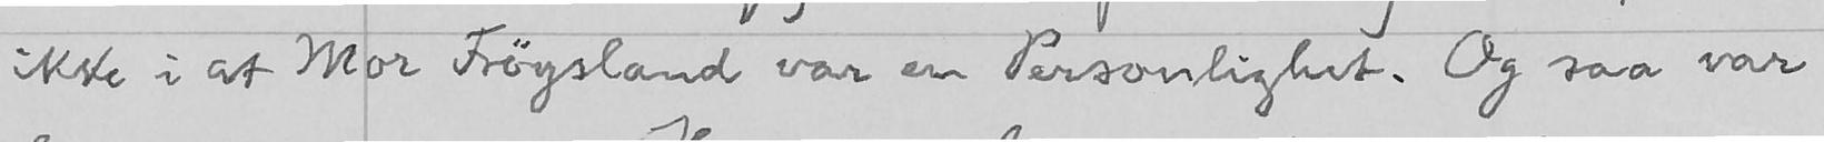ikke i at Mor Frøysland var en Personlighet. Og saa var
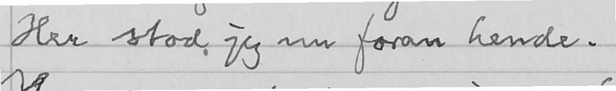Her stod jeg nu foran hende.
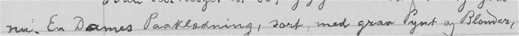nu. En Dames Paaklædning, sort, med graa Pynt og Blonder,
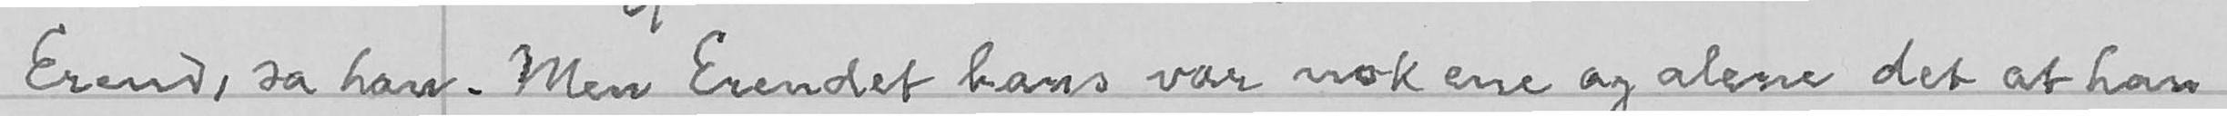Erend, sa han. Men Erendet hans var nok ene og alene det at han
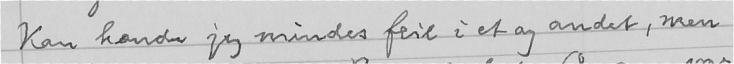Kan hænde jeg mindes feil i et og andet, men
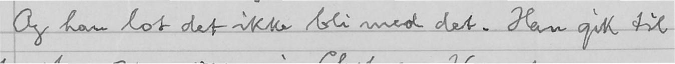Og han lot det ikke bli med det. Han gik til
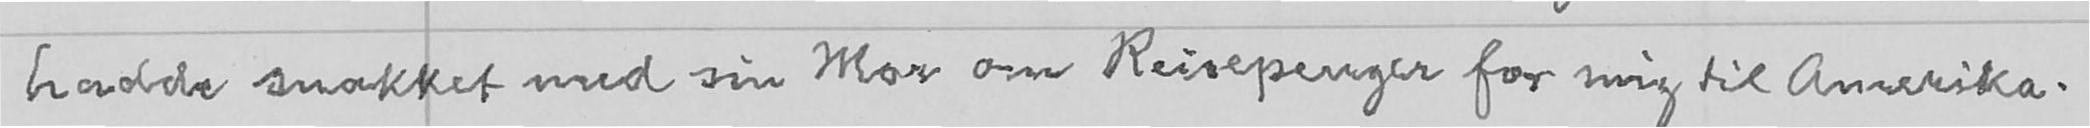hadde snakket med sin Mor om Reisepenger for mig til Amerika.
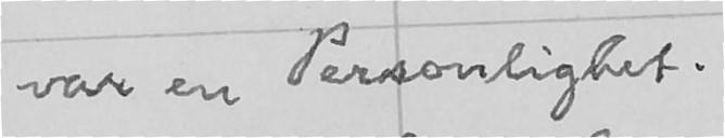var en Personlighet.
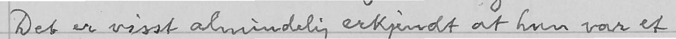Det er visst almindelig erkjendt at hun var et
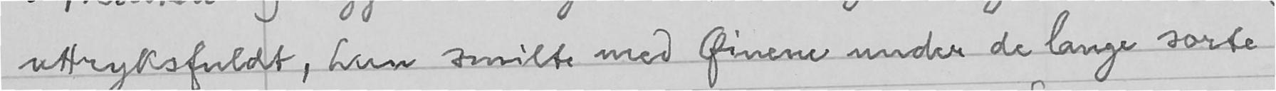uttryksfuldt, hun smilte med Øinene under de lange sorte
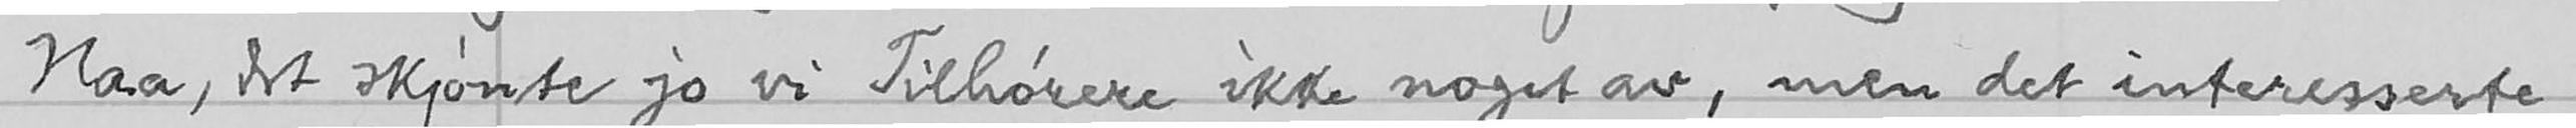Naa, det skjønte jo vi Tilhørere ikke noget av, men det interesserte
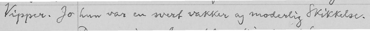Vipper. Jo hun var en svert vakker og moderlig Skikkelse.
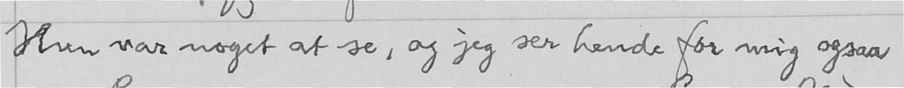Hun var noget at se, og jeg ser hende for mig ogsaa
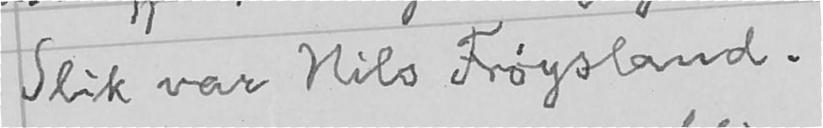Slik var Nils Frøysland.
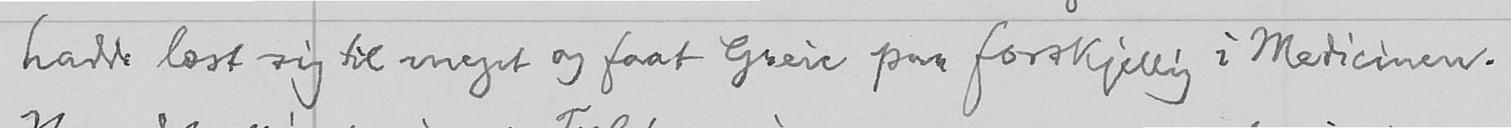hadde læst sig til meget og faat Greie paa forskjellig i Medicinen.
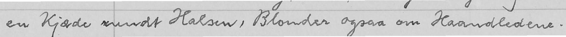en Kjæde rundt Halsen, Blonder ogsaa om Haandledene.
